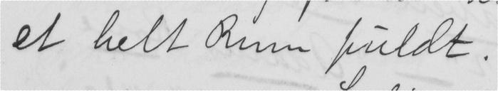et helt Rum fuldt.
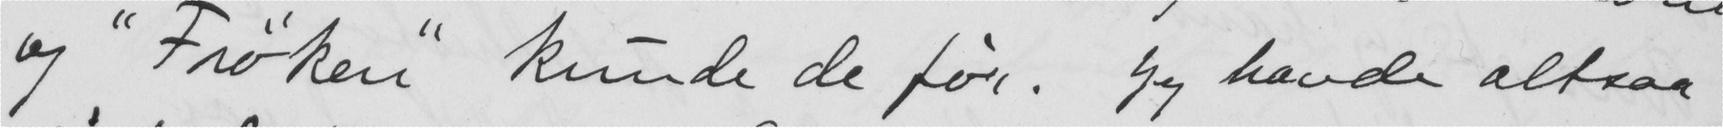og "Frøken" kunde de før. Jeg havde altsaa
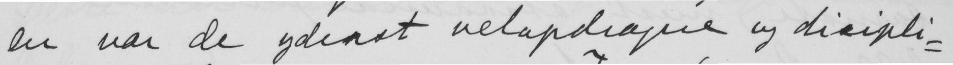en var de yderst velopdragne og disipli-
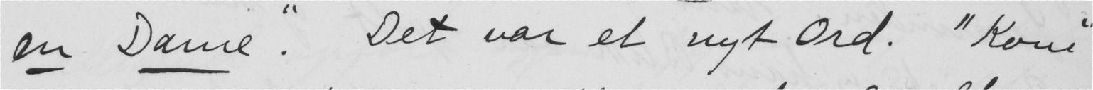en Dame". Det var et nyt Ord. "Kone"
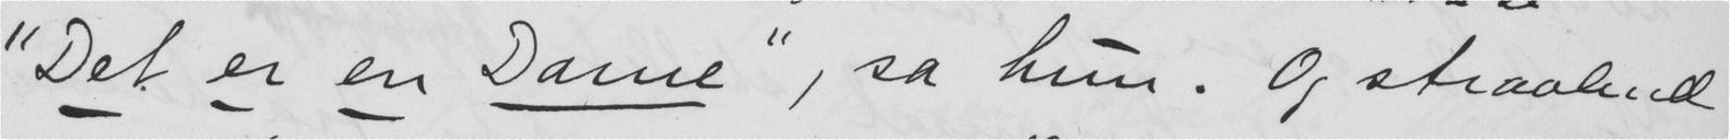"Det er en Dame", sa hun. Og straalend
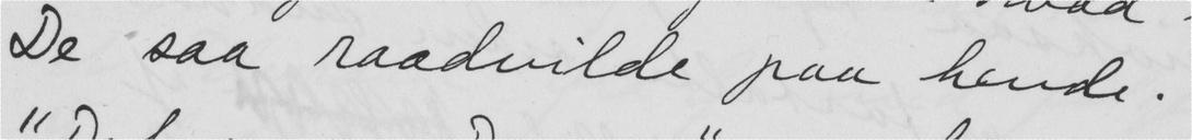De saa raadvilde paa hende.
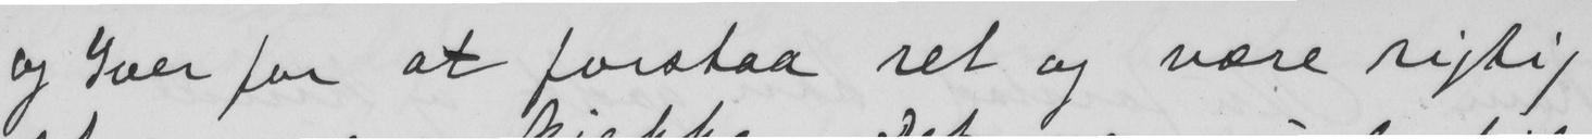og Iver for at forstaa ret og være rigtig
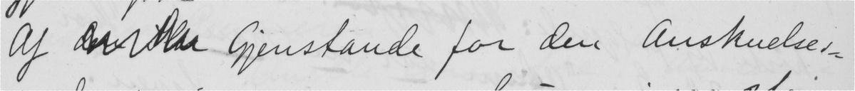Af  Gjenstande for den Anskuelses-
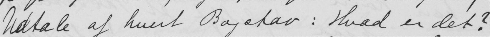Udtale af hvert Bogstav: Hvad er det?
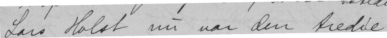Lars Holst nu var den tredie
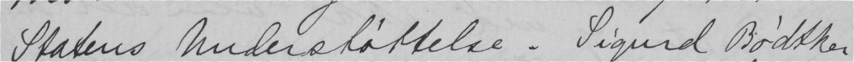Statens understøttelse. Sigurd Bødtker
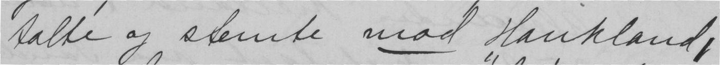talte og stemte mod Haukland,
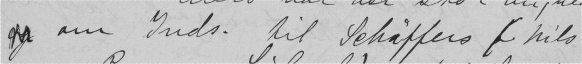om Inds. til Schäffers ( hils
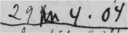29  4.04
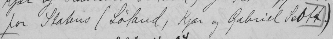for Statens (Løland, Kjær og Gabriel Scott.)
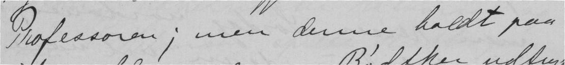Professoren; men denne holdt paa
